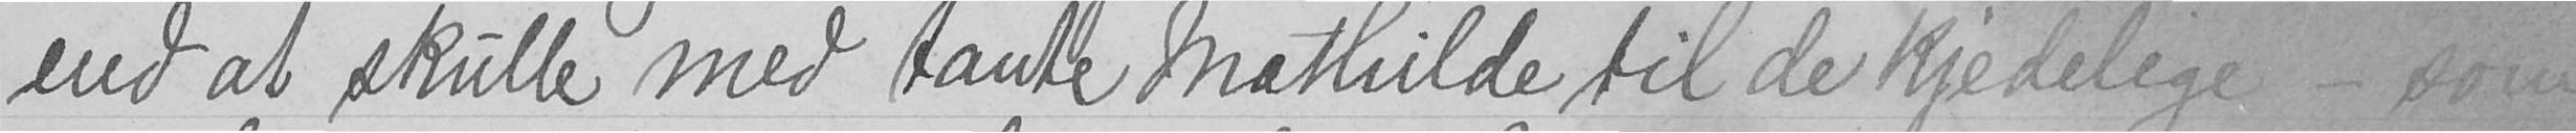end at skulle med tante Mathilde til de kjedelige - som
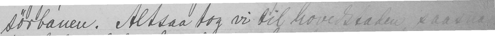sørbanen. Altsaa tog vi til hovedstaden, saa snart
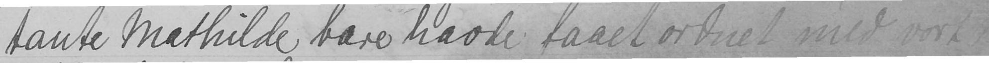tante Mathilde bare havde faaet ordnet med vort
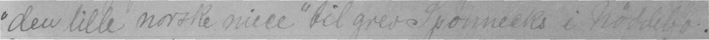"den lille norske niece" til grev Sponneeks i Nøddebo.
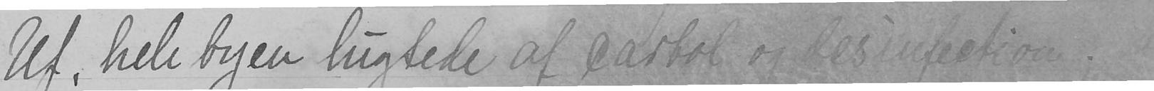Uf, hele byen lugtede af carbol og desinfektion.
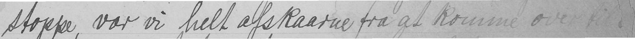stoppe, var vi helt afskaarne fra at komme over til
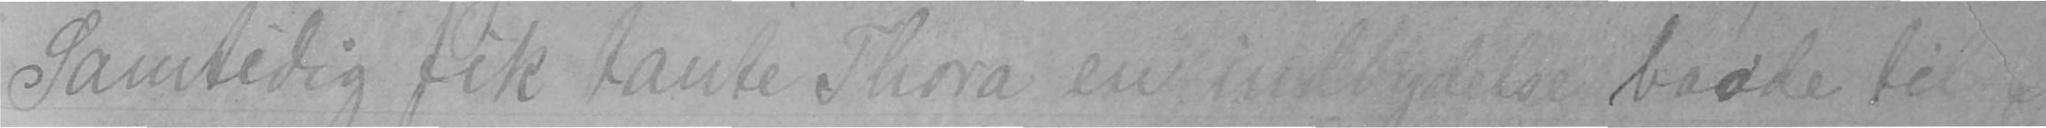Samtidig fik tante Thora en indbydelse baade
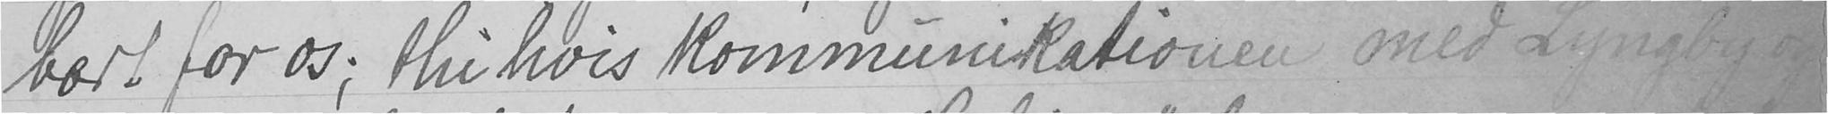bort for os; thi hvis kommunikationen med Lyngby og
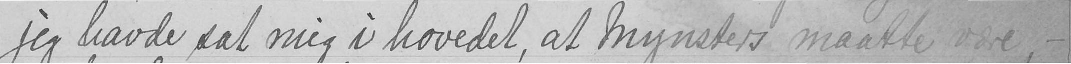jeg havde sat meg i hovedet, at Mynsters maatte være, -
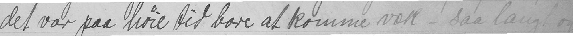det var paa høie tid bare at komme væk - saa langt og
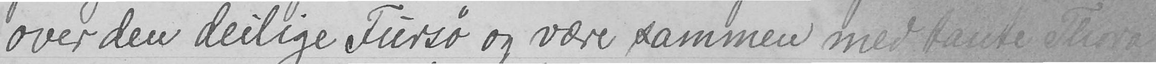over den deilige Fursø og være sammen med tante Thora
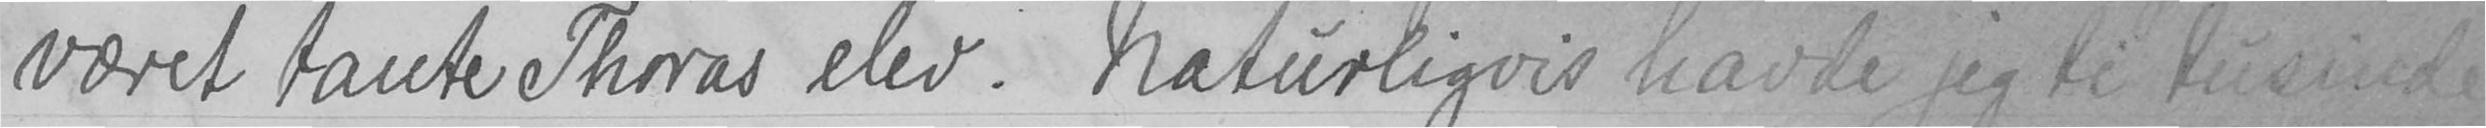været tante Thoras elev. Naturligvis havde jeg ti tusiende
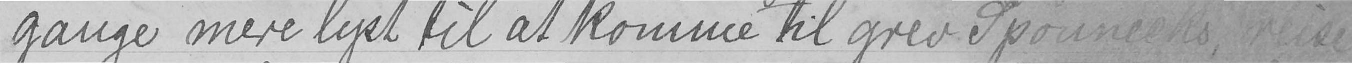gange mere lyst til at komme til grev Sponneeks, reise
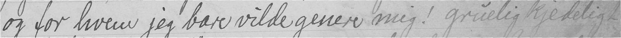og for hvem jeg bare vilde genere mig! gruelig kjedelig
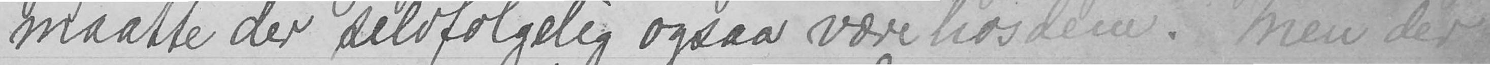maatte der selvfølgelig ogsaa være hos dem. Men der
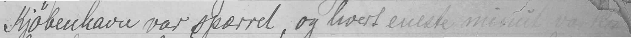Kjøbenhavn var spærret, og hvert eneste minut var taget
